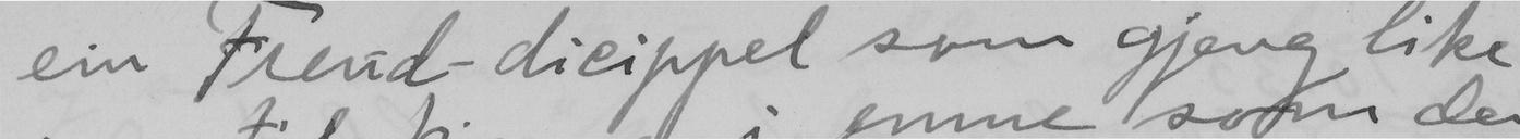ein Freud-dicippel som gjeng like
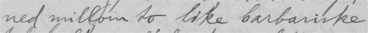ned millom to like barbariske
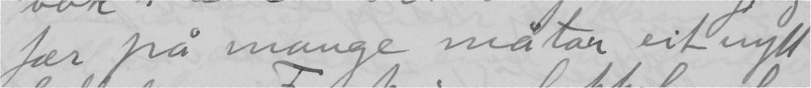fær på mange måtar eit nytt
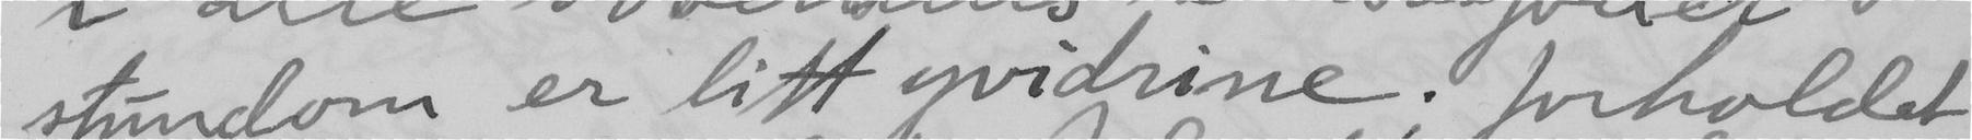stundom er litt yvidrivne; forholdet
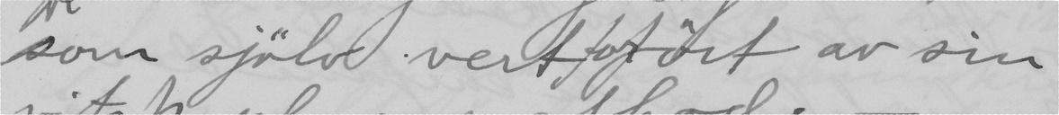som sjölv vert forfört av sin
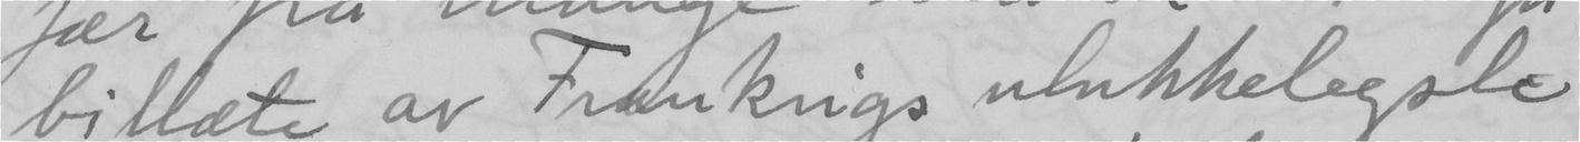billæte av Frankrigs ulukkelegste
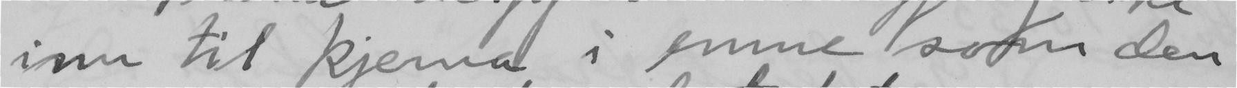inn til kjerna i emne som den
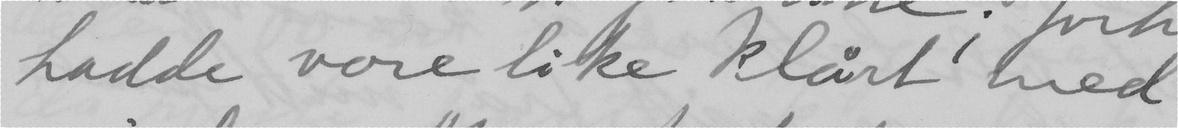hadde vore like klårt med
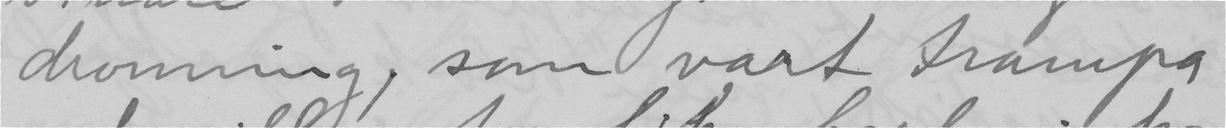dronning, som vart trampa
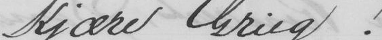Kjære Grieg!
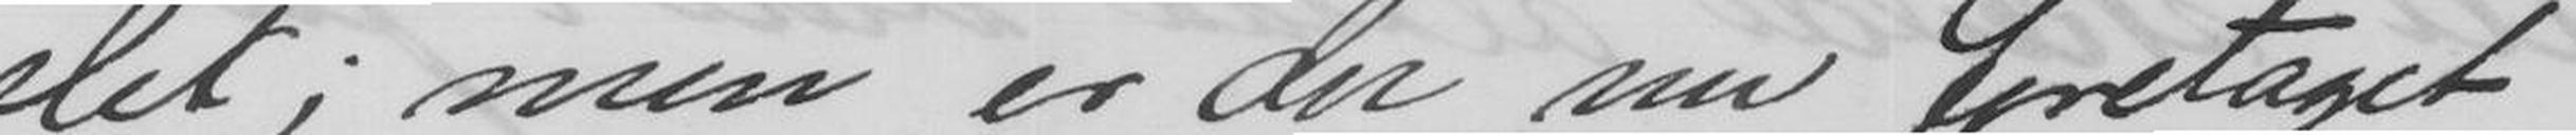slet; men er der nu foretaget
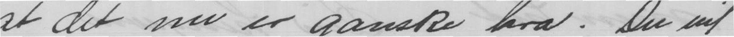at det nu er ganske bra. Du vil
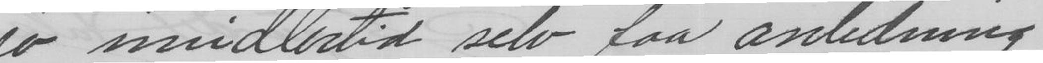jo imidlertid selv faa anledning
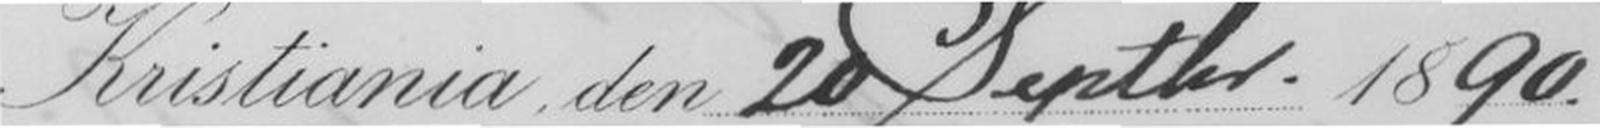Kristiania, den 20 Septbr. 1890
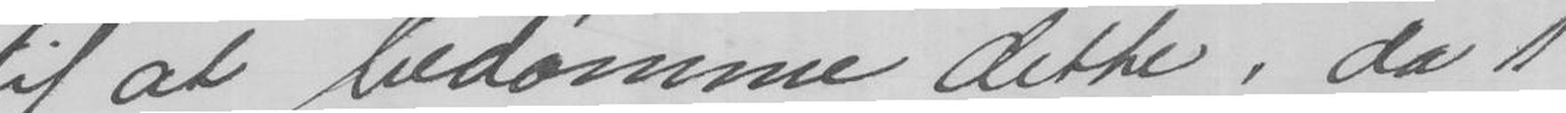til at bedømme dette, da 1
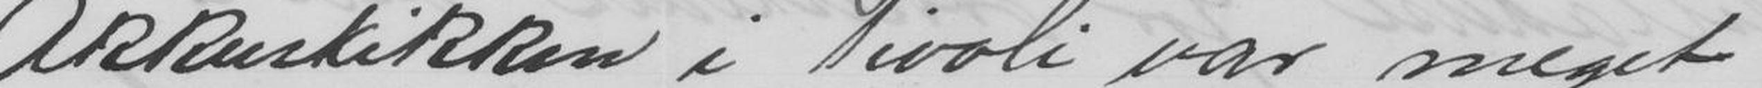Akkustikken i Tivoli var meget
